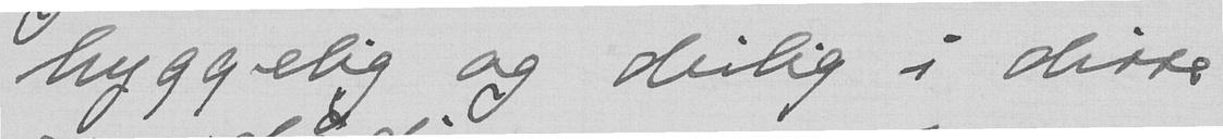hyggelig og deilig i disse
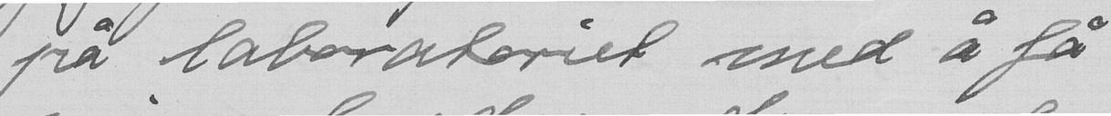på laboratoriet med å få
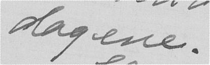dagene.
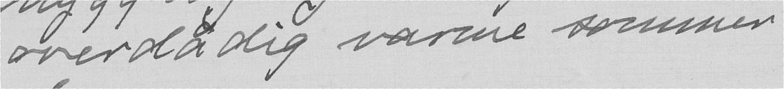overdådig varme sommer
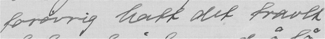jeg forøvrig hatt det travlt
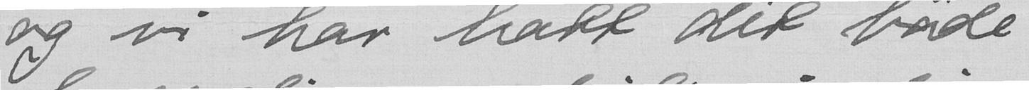og vi har hatt det både
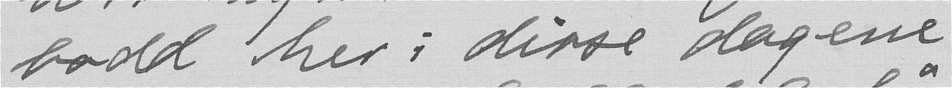bodd her i disse dagene
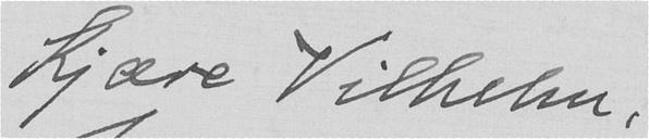Kjære Vilhelm,
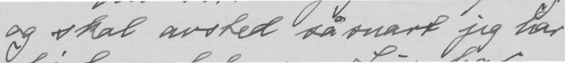og skal avsted så snart jeg har
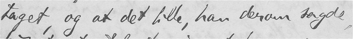taget, og at det lille, han derom sagde,
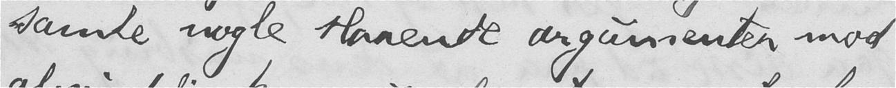samle nogle slaaende argumenter mod
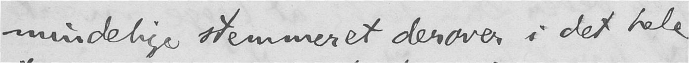mindelige stemmeret derover i det hele
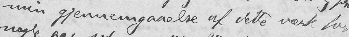min gjennemgaaelse af dette værk for
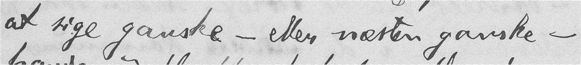at sige ganske – eller næsten ganske -
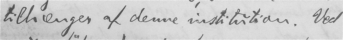tilhænger af denne institution. Ved
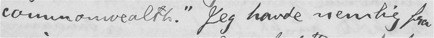commonwealth." Jeg havde nemlig fra
